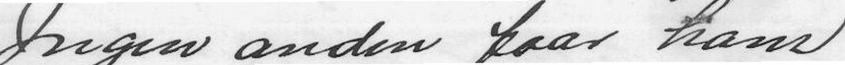Ingen anden faar ham
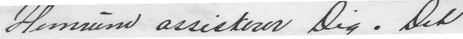Hennum assisterer Dig. Det
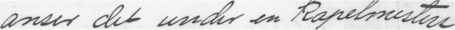anser det under en kapelmesters
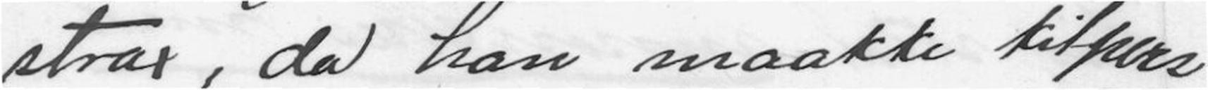strax, da han maatte tilpers,
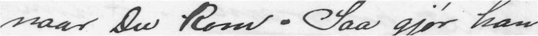naar Du kom. Saa gjør han
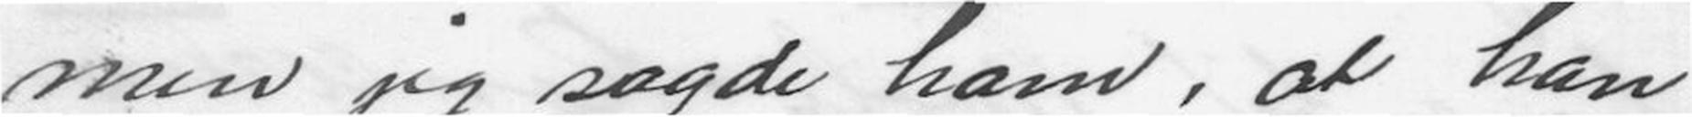men jeg sagde ham, at han
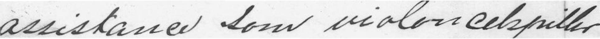assistance som violoncelspiller.
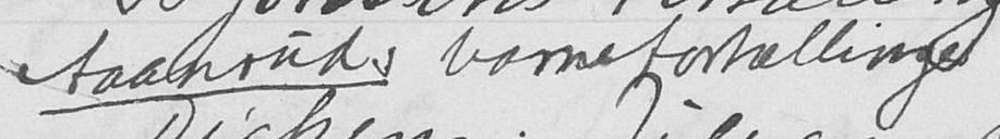Aaanruds barnefortællinger
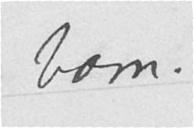barn.
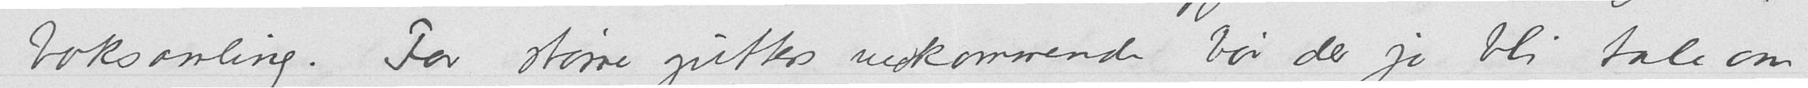boksamling. For større gutters vedkommende bør der jo bli tale om
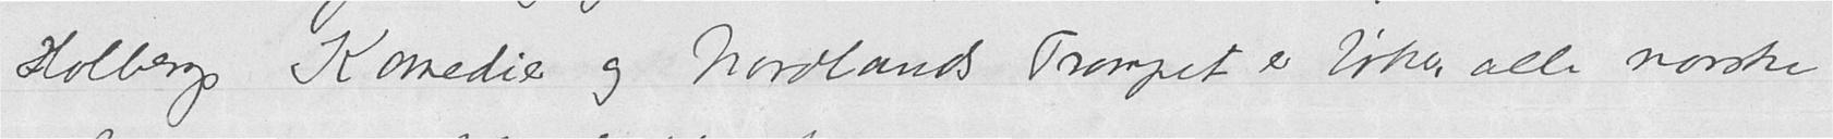Holbergs Komedier og Nordlands Trompet er bøker alle norske
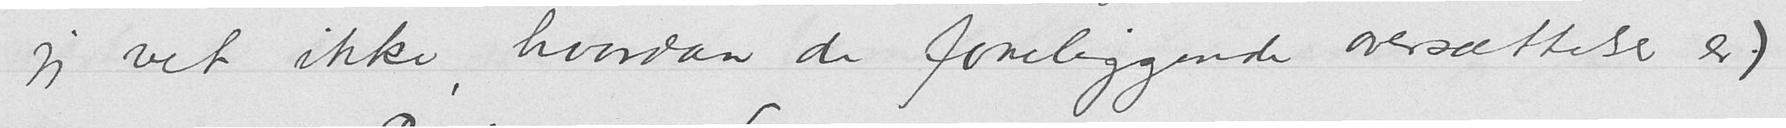jeg vet ikke, hvordan de foreliggende oversættelser er)
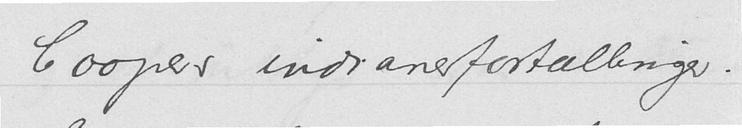Coopers indianerfortællinger.
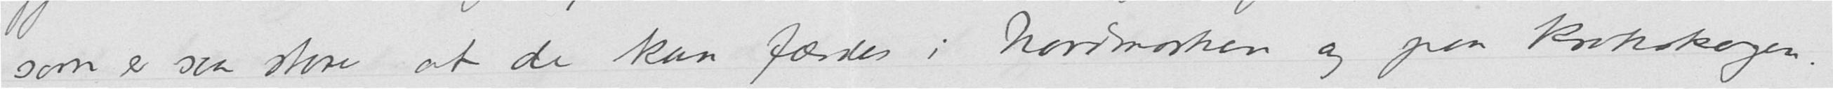som er saa store at de kan færdes i Nordmarken og paa Krokskogen,
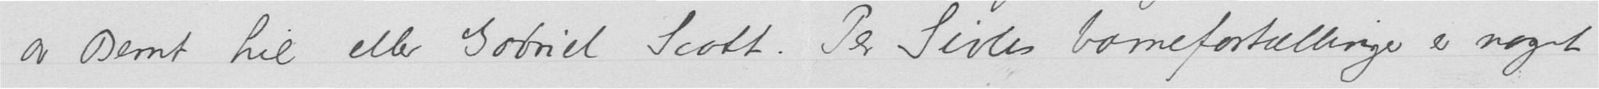av Bernt Lie eller Gabriel Scott. Per Sivles barnefortællinger er noget
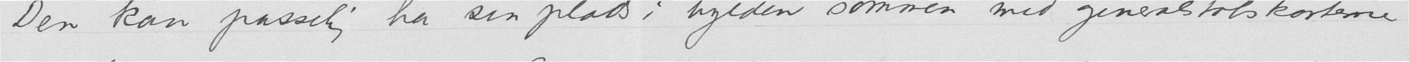Den kan passelig ha sin plads i hylden sammen med generalstabskarterne
